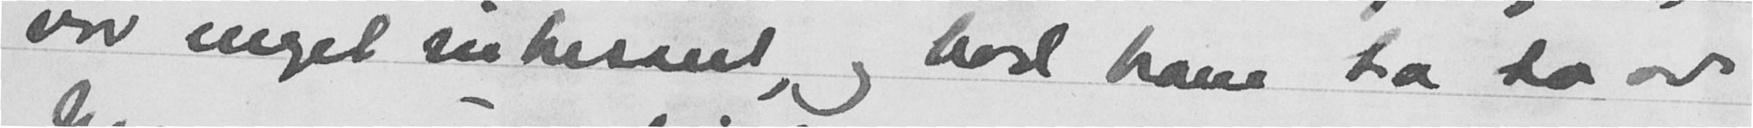var meget intresert, og bød ham ta to av
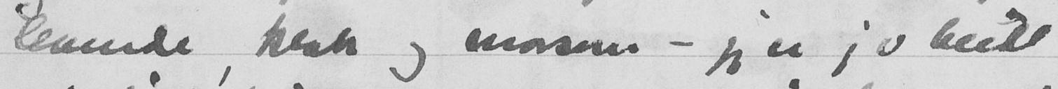Levende, klok og morsom - jeg er jo blitt
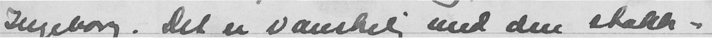Ingeborg. Det er vanskelig med den stokk-
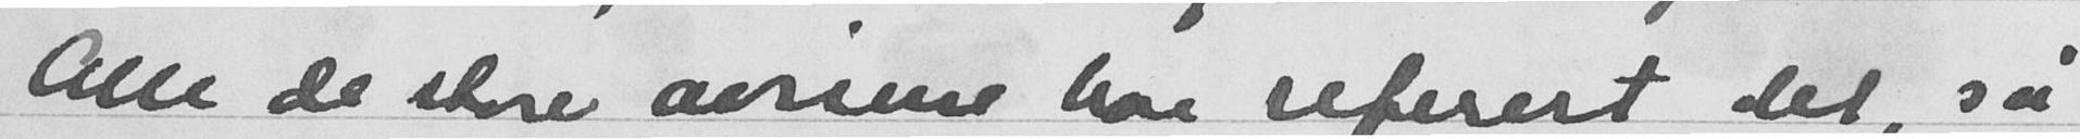Alle de store avisen har referert det, så
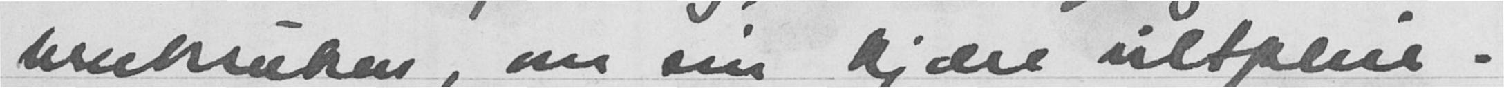bruksuken, om sin kjære viltpleie.
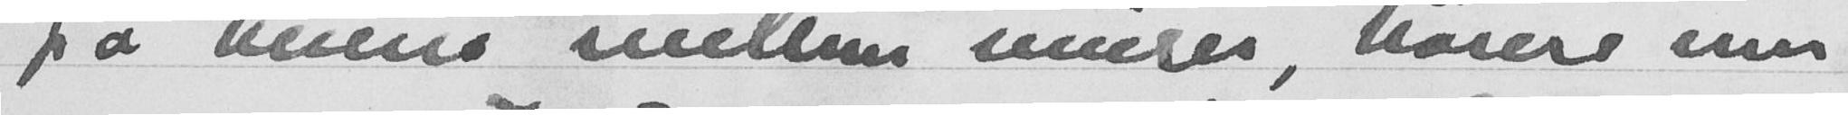på veiene mellem murer, høyere enn
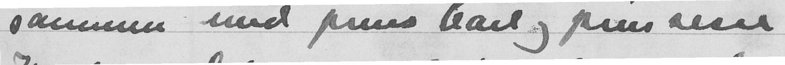sammen med prins Carl og prinsesse
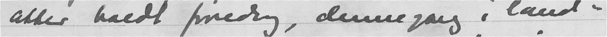atter holdt foredrag, denne gang i land-
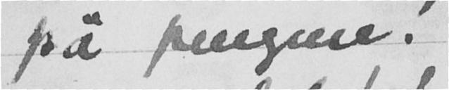på pengene!
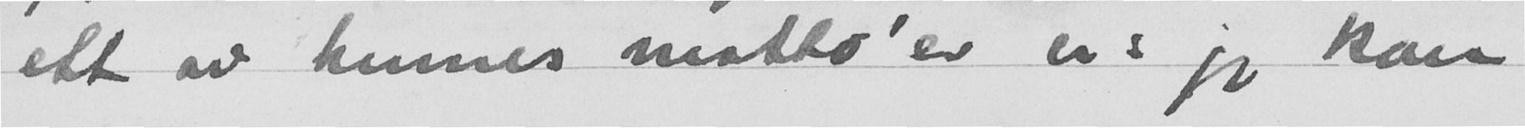ett av hennes motto'er er: jeg kan
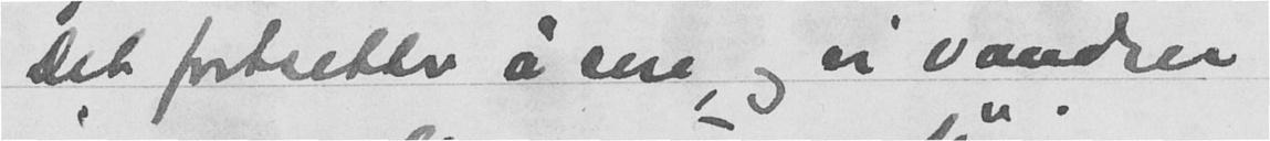Det fortsetter å sne og vi vandrer
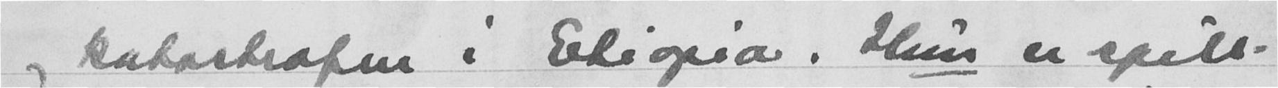og katastrofen i Etiopia. Hun er spilt.
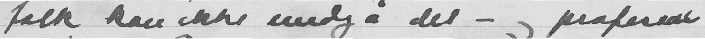folk kan ikke undgå det - og professor
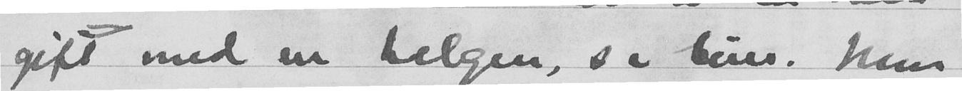gift med en helgen, sa hun. Men
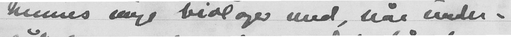hennes unge biologer med, når under-
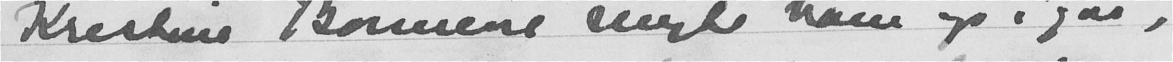Kristine Bonnevie ringte ham op i går,
